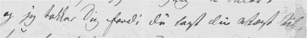og jeg takker Dig fordi Du lagt Din Vægt til,
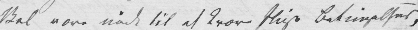skal være nødt til at kræve slige Betingelser,
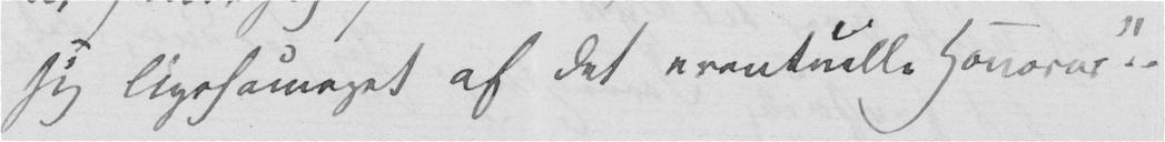sig ligesåmeget af det eventuelle Honorar"..
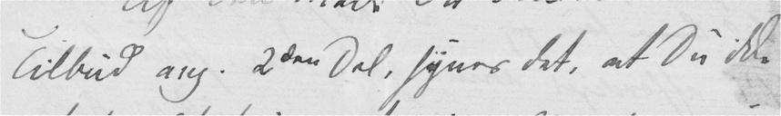Tilbud ang. 2den Del, synes det, at Du ikke
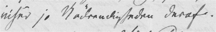viser jo Nødvendigheden deraf.
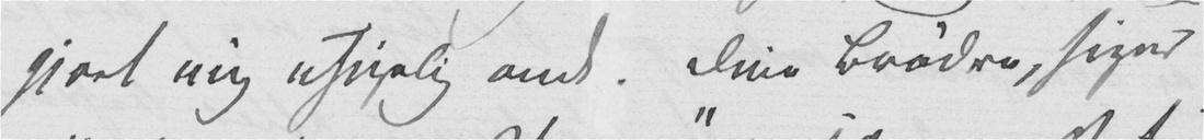gjort mig usigelig ondt. Dine Brødre, siger
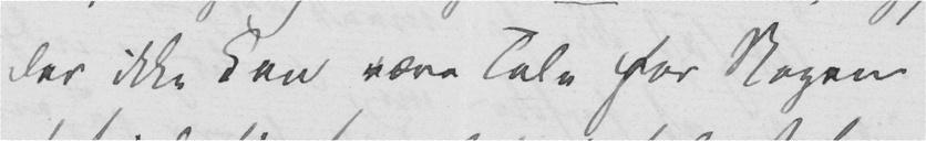der ikke kan være Tale for Nogen
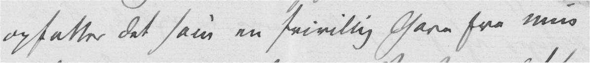opfatter det som en frivillig Gave fra min
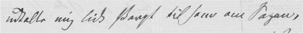udtalte mig lidt skarpt til ham om Sagen,
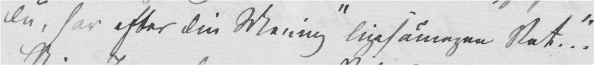Du, har efter din Mening "ligesåmegen Ret..."
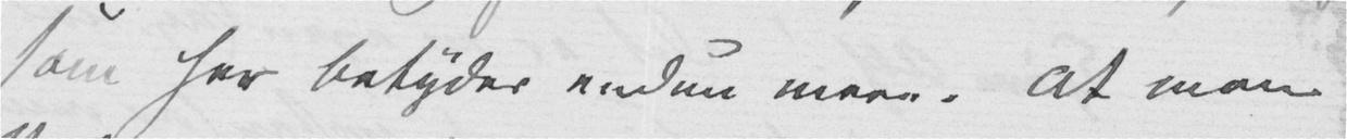som her betyder endnu mere. At man
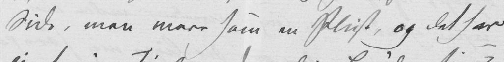Side, men mere som en Pligt, og det har
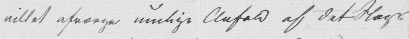villet afværge mulige Anfald af det Slags
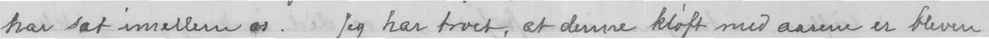har sat imellem os. Jeg har troet, at denne kløft med aarene er bleven
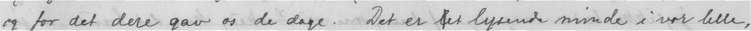og for det dere gav os de dage. Det er et lysende minde i vor lille,
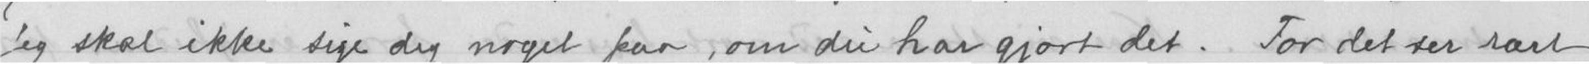Jeg skal ikke sige dig noget paa, om du har gjort det. For det ser rart
